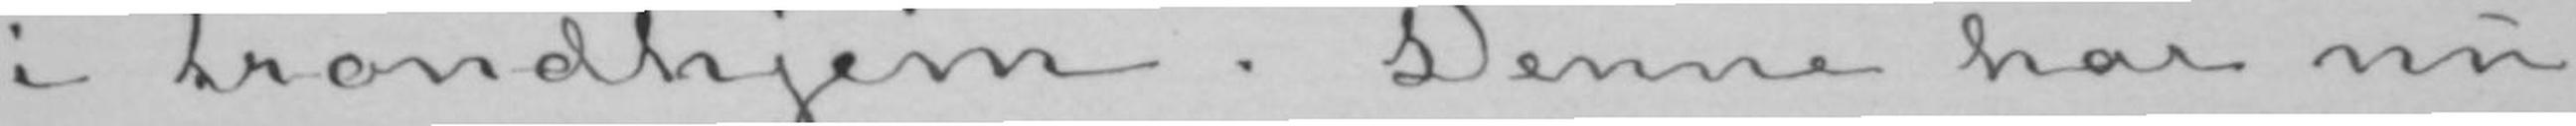i Trondhjem. Denne har nu
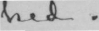hed.
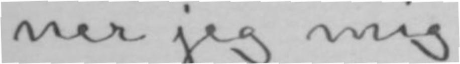ner jeg mig
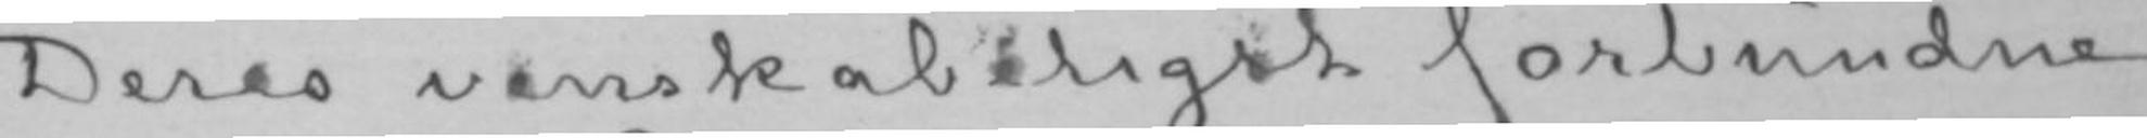Deres venskabeligst forbundne
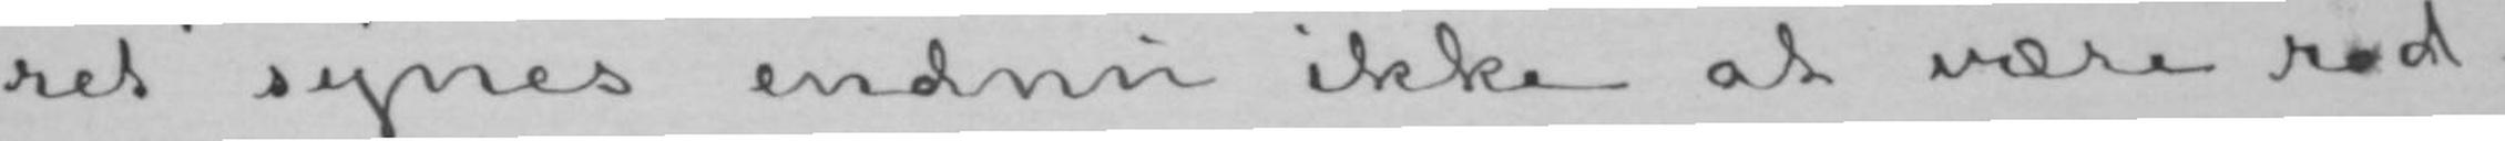ret synes endnu ikke at være rod-
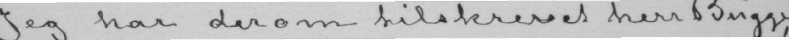Jeg har derom tilskrevet herr Bugge,
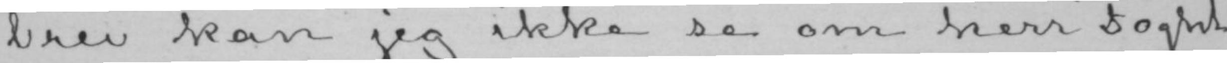brev kan jeg ikke se om herr Foght
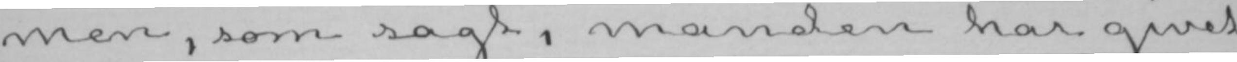men, som sagt, manden har givet
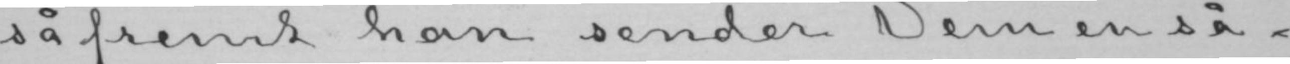såfremt han sender Dem en så-
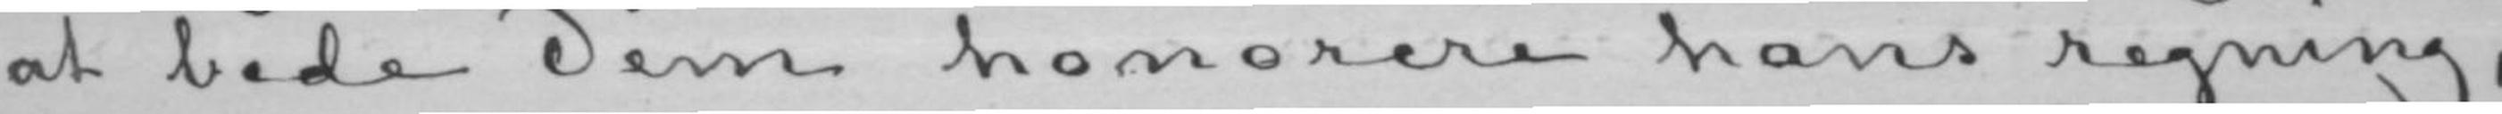at bede Dem honorere hans regning,
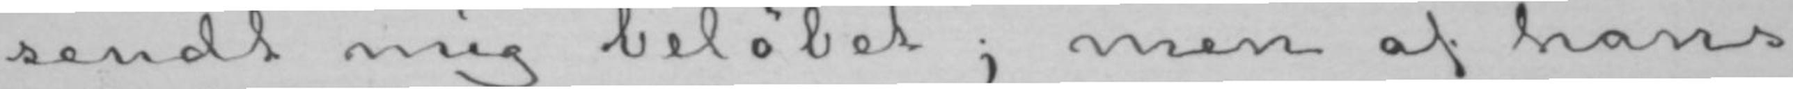sendt mig beløbet; men af hans
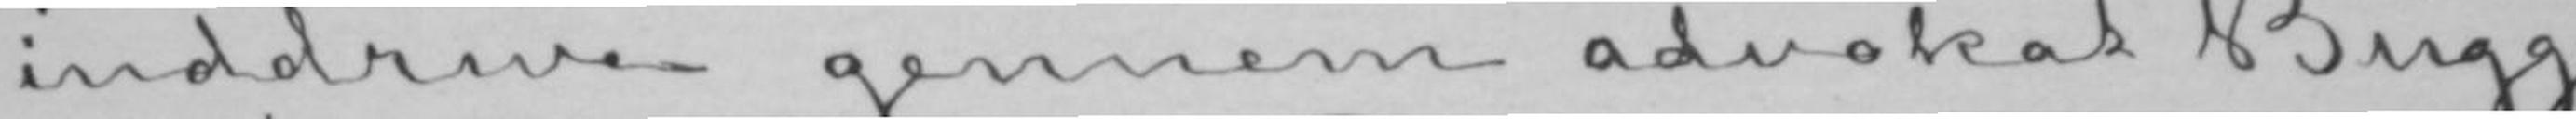inddrive gennem advokat Bugge
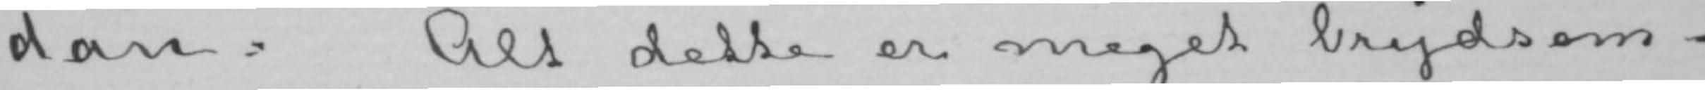dan. Alt dette er meget brydsom-
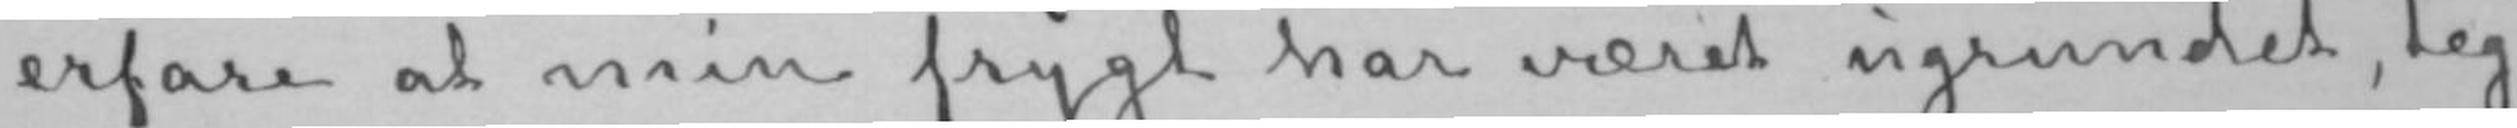erfare at min frygt har været ugrundet, teg-
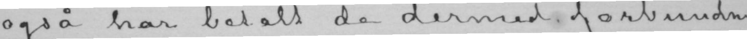også har betalt de dermed forbundne
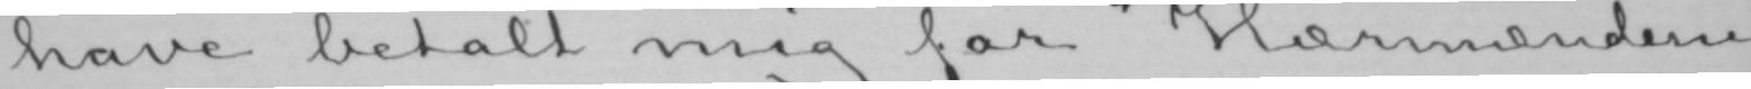have betalt mig for «Hærmændene
